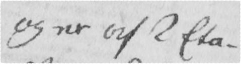og er af 2 Eta-
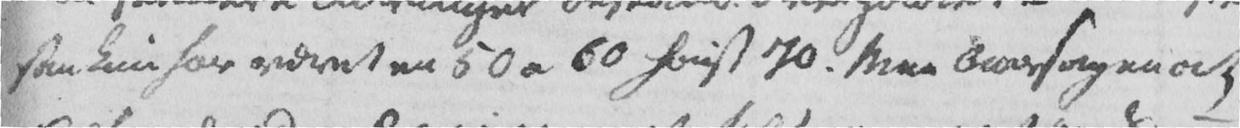som kun har været en 50 a 60 høist 70. Men Aarsagen at
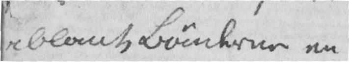iblant Bønderne en
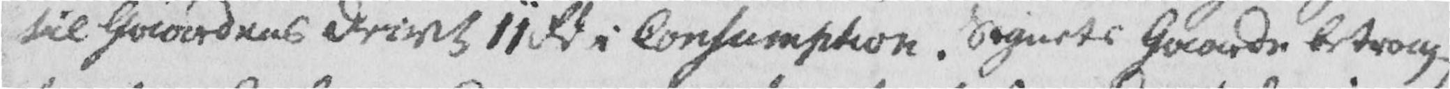til Gaardens drivt 11 Rd i Consumption. Sognets Gaarde betrag-
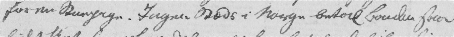for en Stuepige. Ingen Stæd i Norge betal Bonden saa
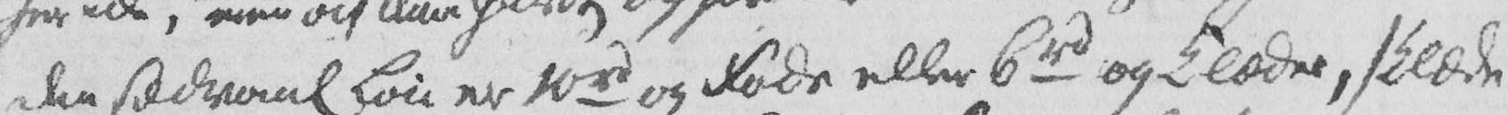den sædvanl Løn er 10 rd og Føde eller 6 rd og Klæder, / Klæde
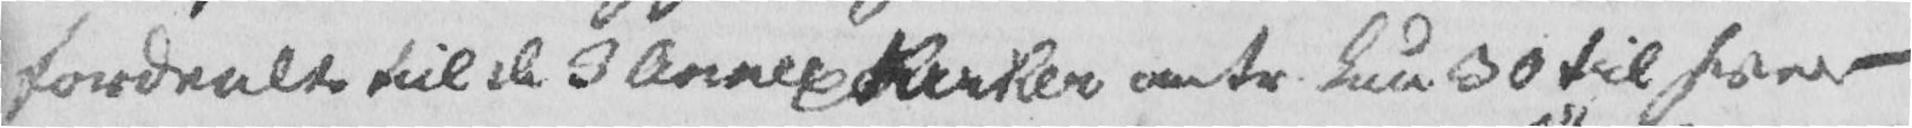fordeelt til de 3 Annex Kirker omtr. kun 30 til hver -
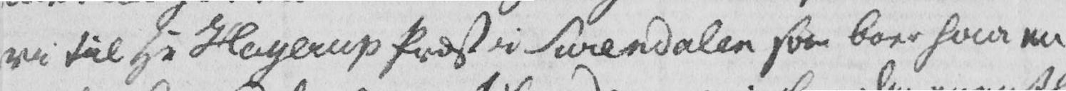vi til Hr Hagerup Præst i Surendalen som boer paa en
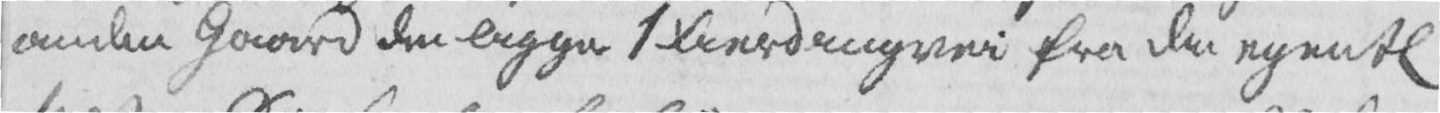anden Gaard den ligge 1 Fierdingvei fra den egentl
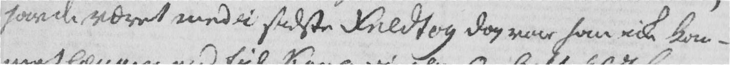havde været med i sidste Feldtog dog var han icke kom-
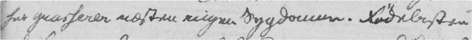her grasserer næsten ingen Sygdomme. Fødelisten
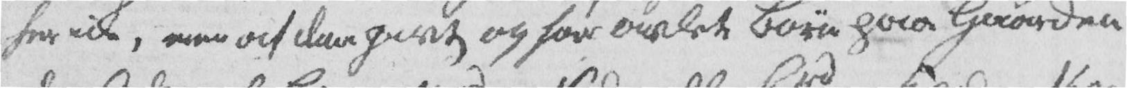her ikke, een af dem givt og har avlet Børn paa Gaarden
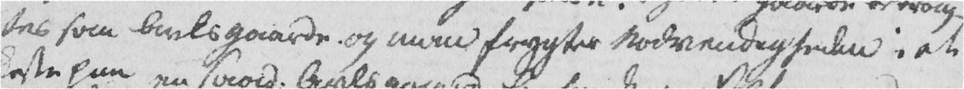tes som Avlsgaarde og man frygter Nødvendigheden i at
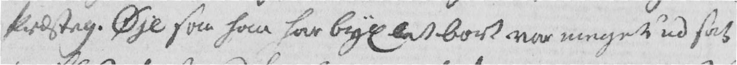Præsteg. Øje som han har byxlet bort var meget udsat
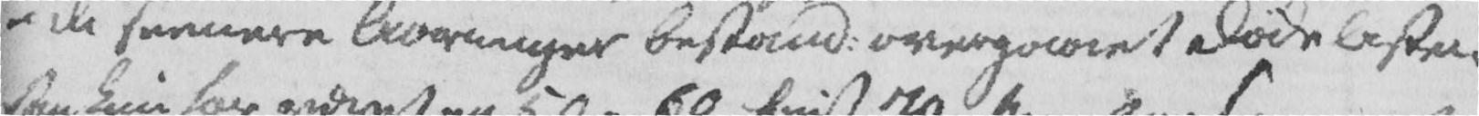de seenere Aaringer bestand. overgaaet Dødebestn.
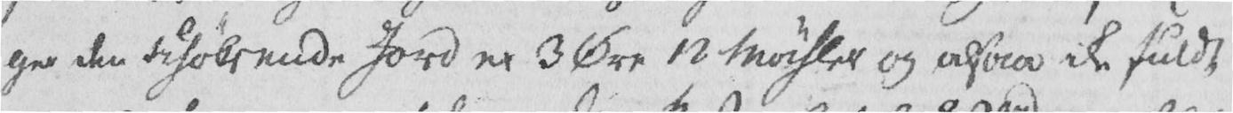ger den tilhørende Jord er 3 Øre 12 Møihler og altsaa icke fuldt
